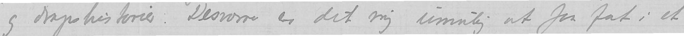og drapshistorier. Desværre er det mig umulig at faa fat i et
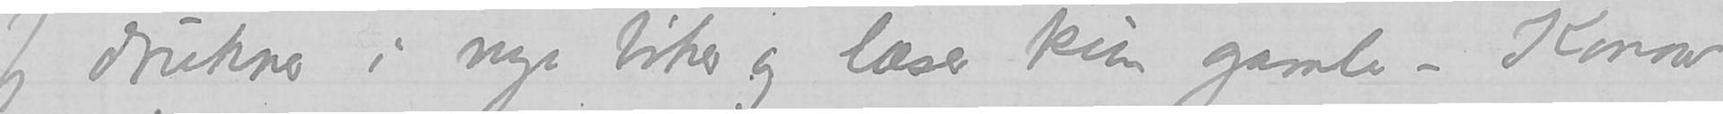Jeg drukner i nye bøker og læser kun gamle – Konow
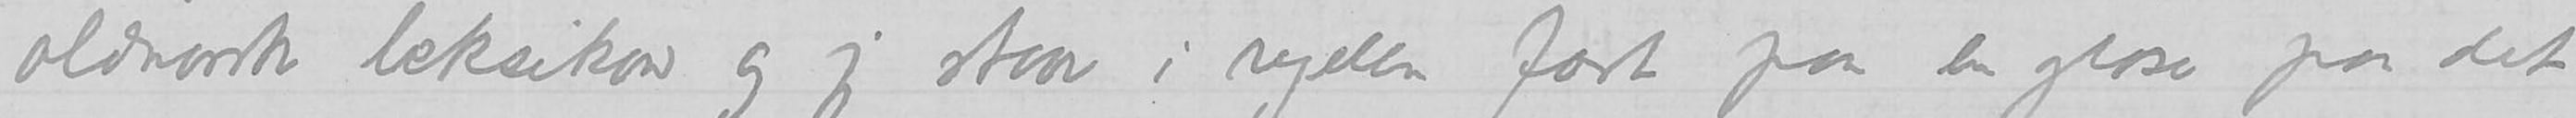oldnorsk leksikon og jeg staar i regelen fast paa en glose paa det
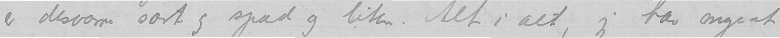er desværre sart og spæd og liten.  Alt i alt, jeg har mye at
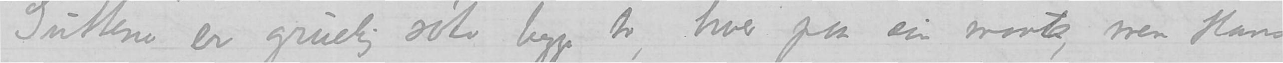Guttene er grundig søte begge to, hver paa sin maate, men Hans
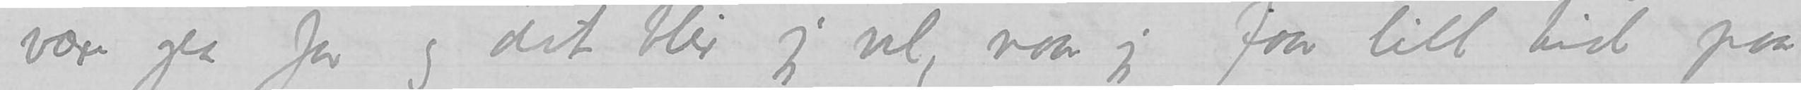være gla for og det blir jeg vel, naar jeg faar litt tid paa
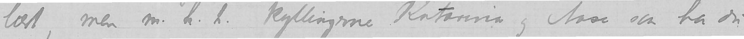læst, men m.h.t. kyllingerne Katarina og Aase saa har du
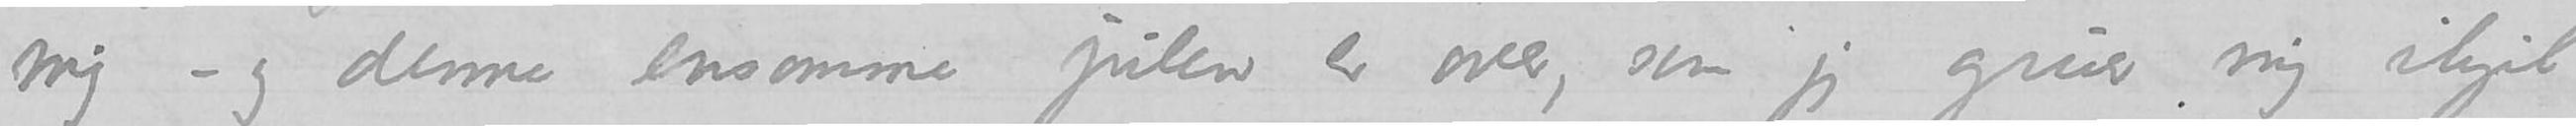mig – og denne ensomme julen er over, som jeg gruer mig ihjel
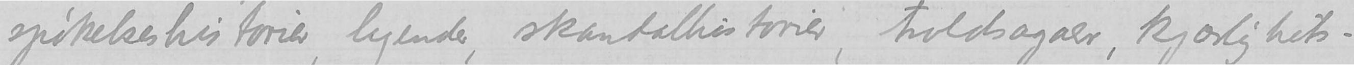spøkelseshistorier, legender, skandalhistorier, troldsagaer, kjærlighets-
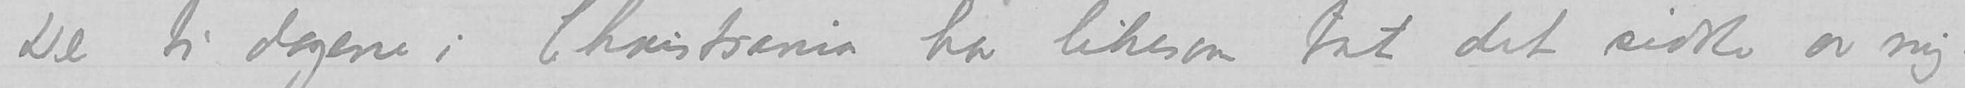De ti dagene i Christiania har likesom tat det sidste av mig.
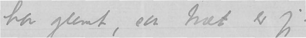har glemt, saa træt er jeg.
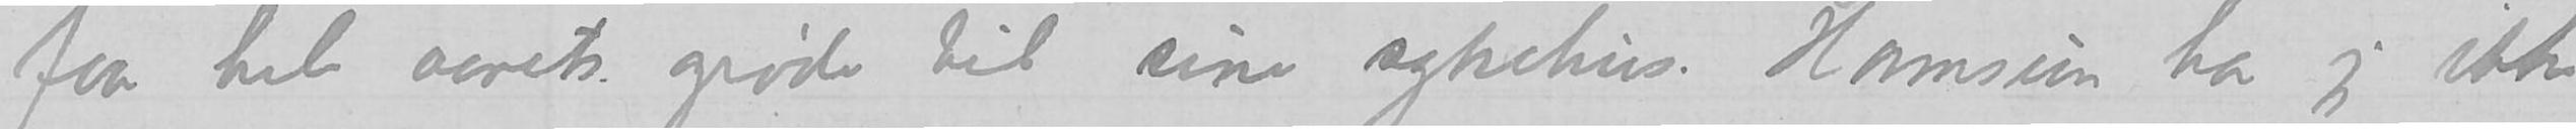faar hele aarets grøde til sine sykehus.  Hamsun har jeg ikke
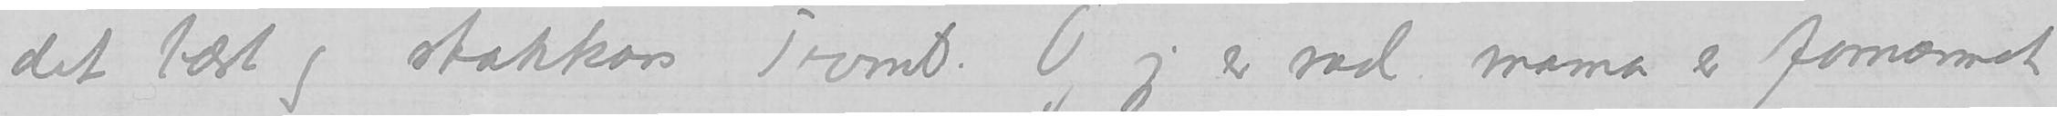det bæst og stakkars Trond. Og jeg er ræd mama er fornærmet
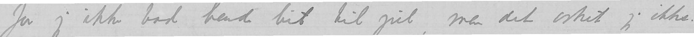for jeg ikke bad hende hit til jul, men det orket jeg ikke.
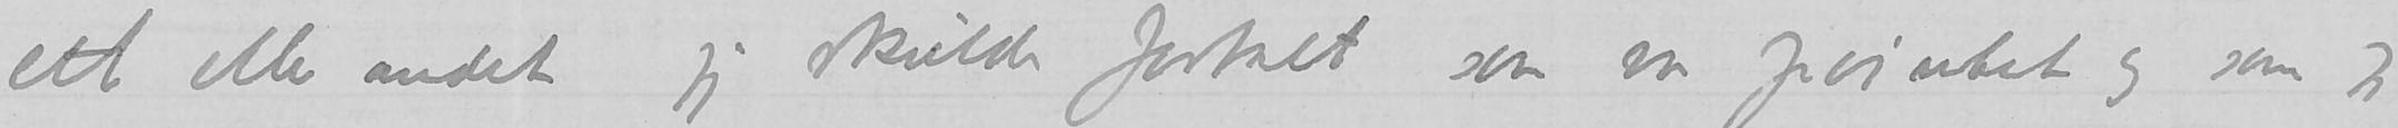ett eller andet jeg skulde fortalt som var pointet og som jeg
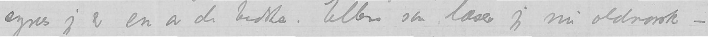synes jeg er en av de bedste.  Ellers saa læser jeg nu oldnorsk –
